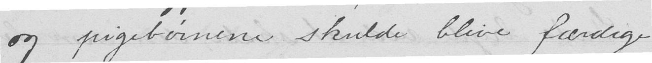og pigebørnene skulde blive færdige
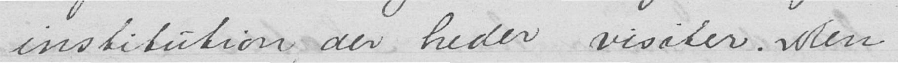institution, der heder visiter. Men
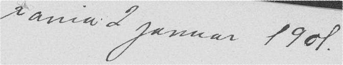Xania 2 januar 1901.
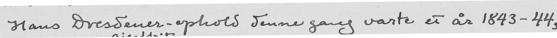Hans Dresdener-ophold denne gang varte et år 1843-44,
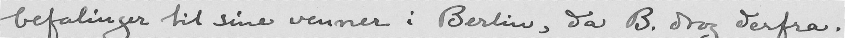befalinger til sine venner i Berlin, da B. drog derfra.
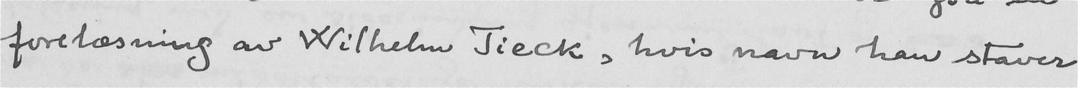forelæsning av Wilhelm Tieck, hvis navn han staver
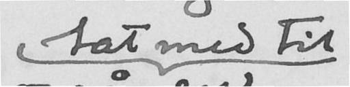tat med til
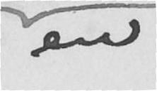en
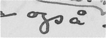også
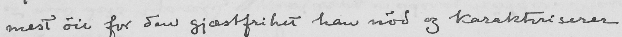mest øie for den gjæstfrihet han nød og karakteriserer
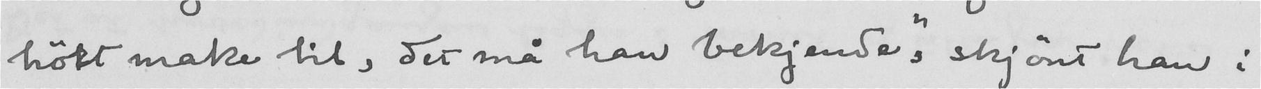hørt make til, det må han bekjendes, "skjønt han i
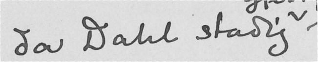da Dahl stadig.
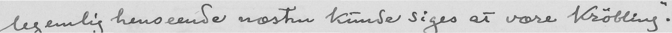legemlig henseende næsten kunde siges at være krøbling."
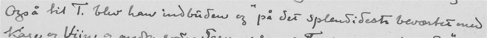Også til T. blev han indbuden og "på det splendideste beværtet med
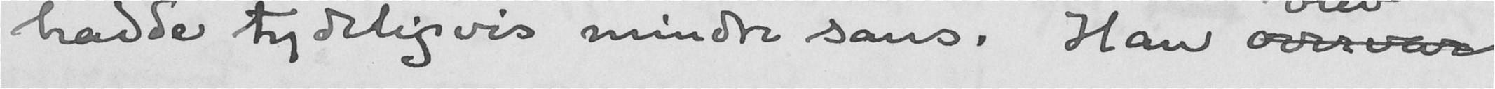hadde tydeligsvis mindre sans. Han overvar
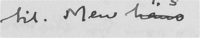til. Men hans
